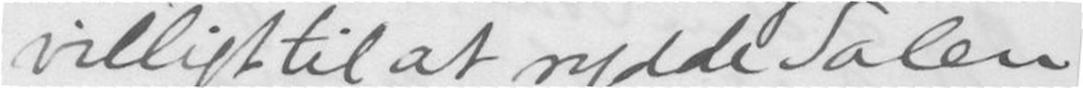villigt til at rydde Salen
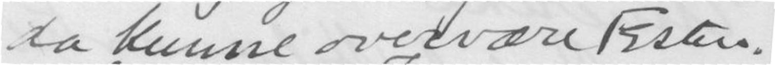da kunne overvære Festen.
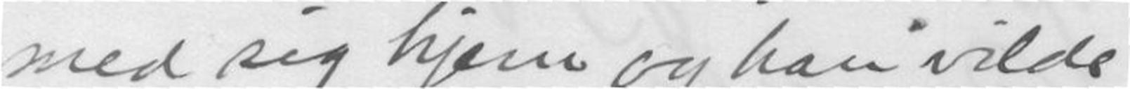med sig hjem og han vilde
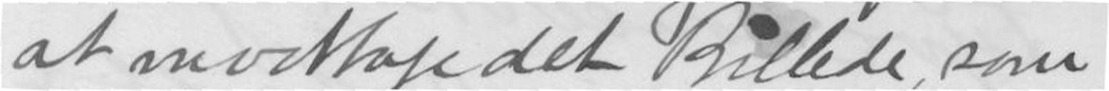at modtage det Billede, som
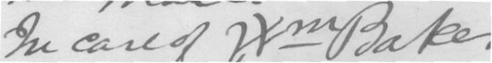In care of Wm Baker
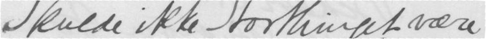Skulde ikke Storthinget være
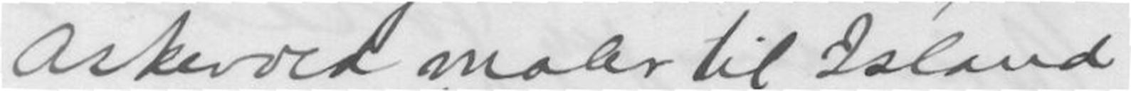Askevold maler til Island
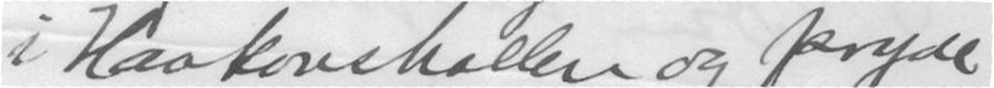i Haakonshallen og pryde
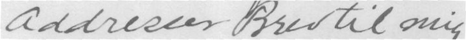Addresser Brev til mig
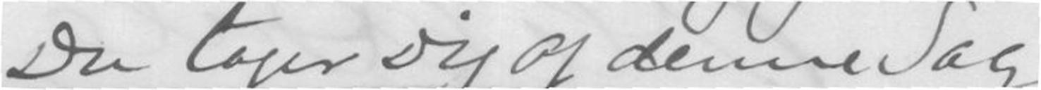Du tager Dig af denne Sag
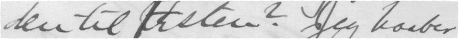den til Festen? Jeg haaber
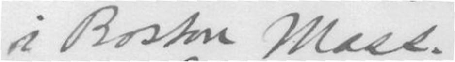i Boston Mass.
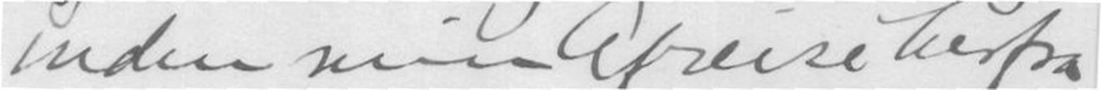inden min Afreise herfra
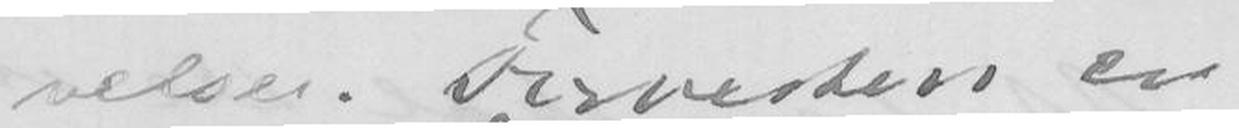velse. Foresten er
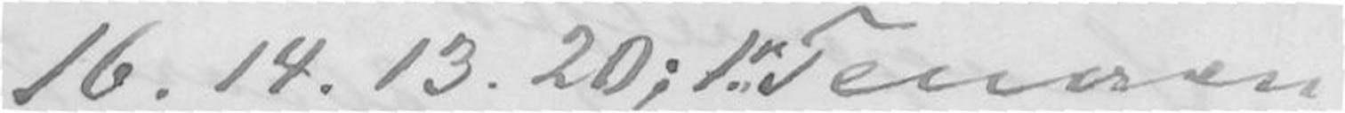16. 14. 13. 20; 1ste Tenoren
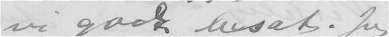vi godt besat. Jeg
